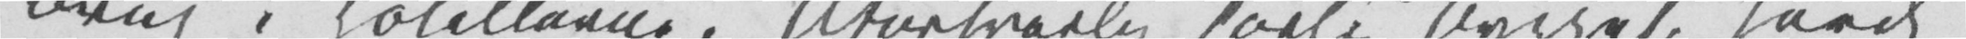Brug i Hotellerne. Uforsvarlig farlig bygget, havde
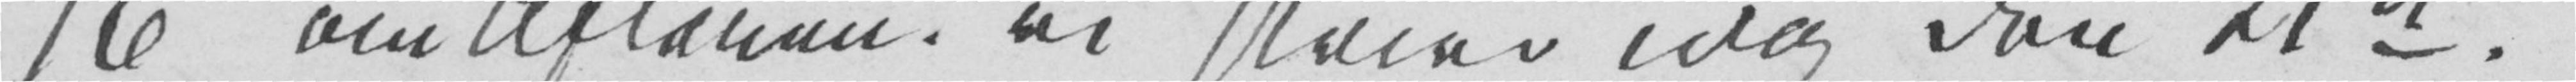16de om Aftenen, vi skriver idag den 21de.
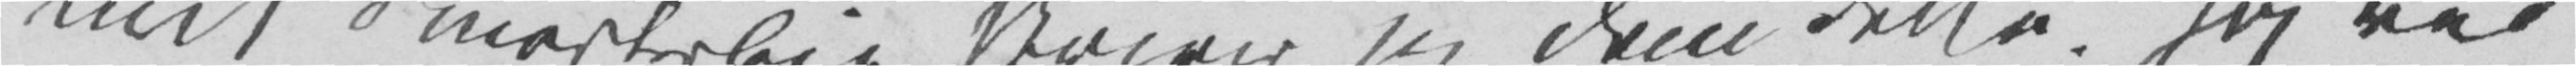mit Smertesleie skriver jeg Dem dette. Jeg ved
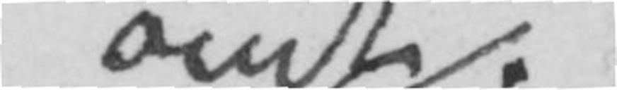ondt
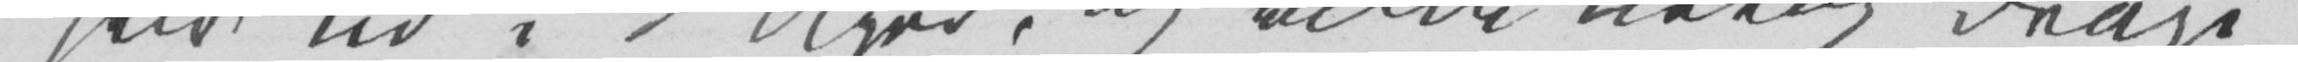selv ud i 3 Uger, og vilde netop drage
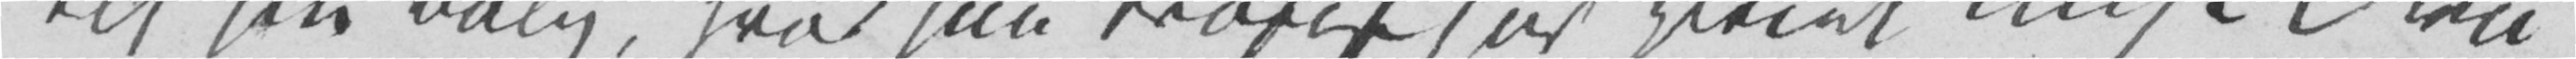til sin Bolig, hvor hun trofast har pleiet mig. Fra
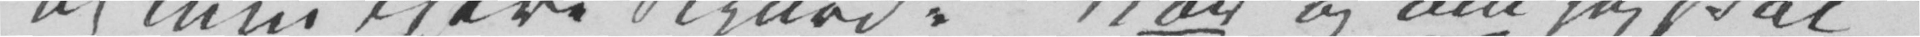og min kjere Sigurd. Når og om jeg skal
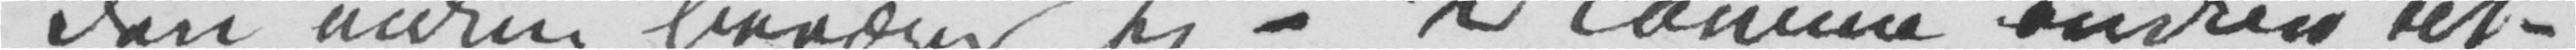den endnu bevæger sig – ½ Tomme endnu til –
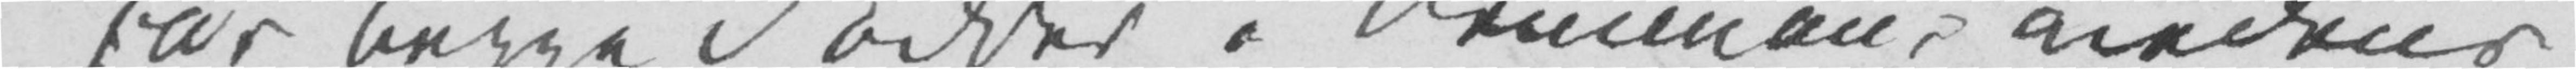får begge Fødder i Klemmen medens
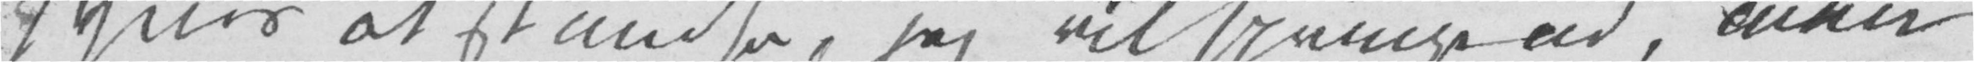synes at standse, jeg vil springe ud, men
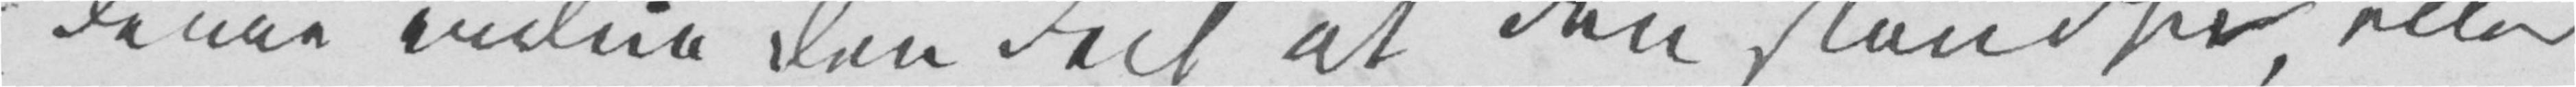denne endnu den Feil at den standser, eller
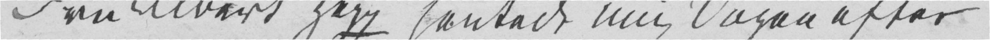Fru Albert Hepp hentede mig Dagen efter
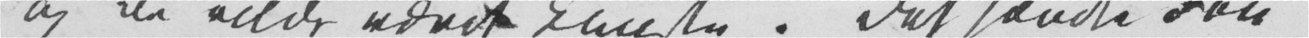og de vilde været knuste. Det hændte den
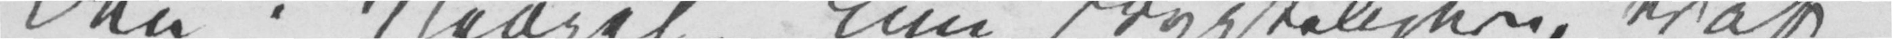den i Neapel, kun frygteligere, traf
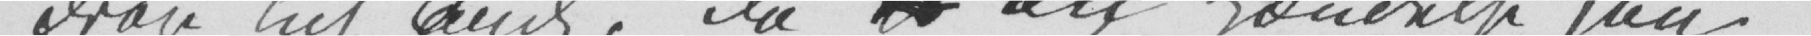drage lidt Ånde, da et ny Hændelse som
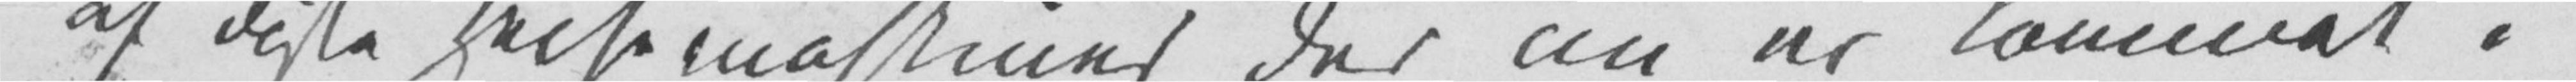af disse Heisemaskiner der nu er kommet i
